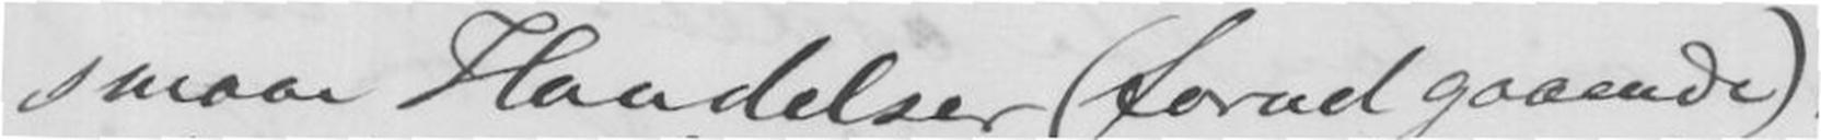smaae Hæmdelser (forud gaaende)
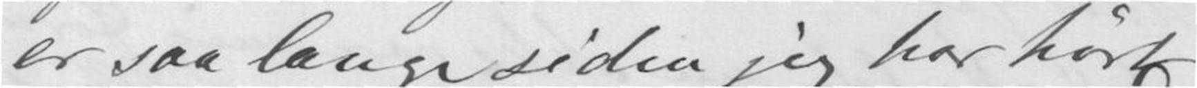er saa længe siden jeg har hørt
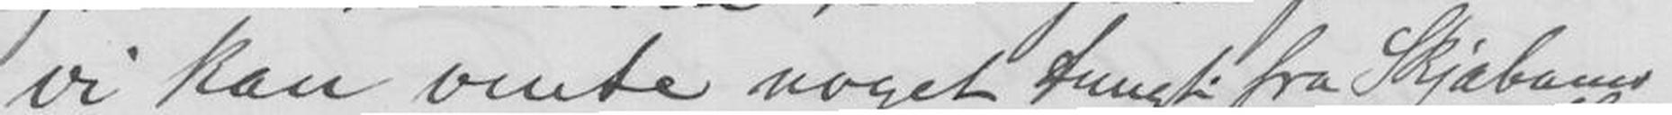vi kan vente noget tungt fra Skjæbnens
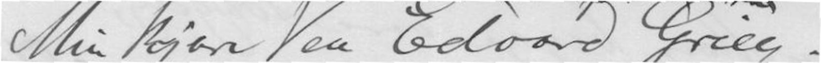Min kjære Ven Edvard Grieg.
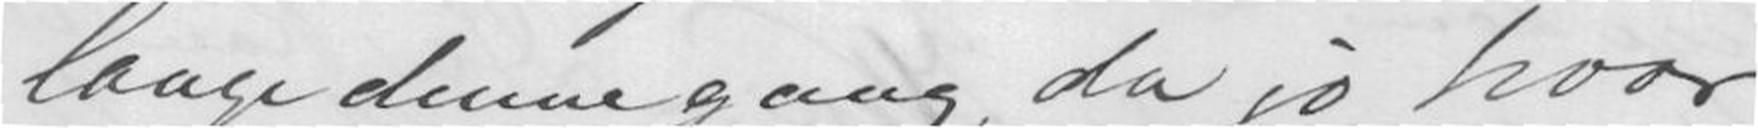længe denne gang, da jo hvor
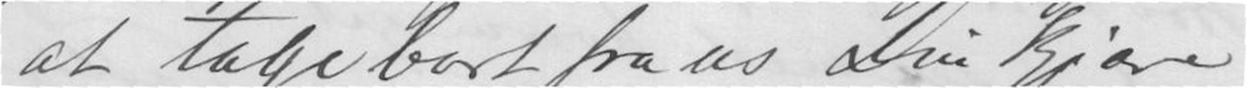at tage bort fra os Din Kjære
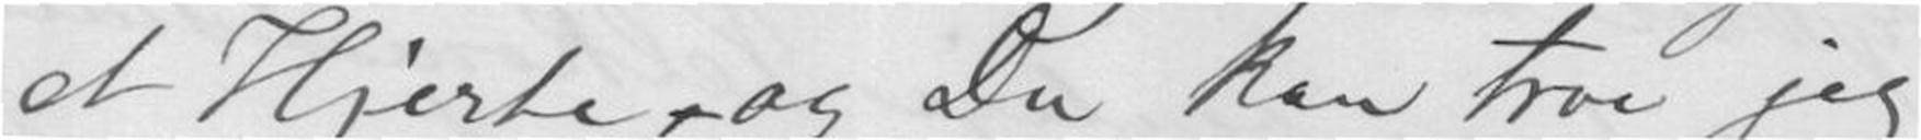et Hjerte, og Du kan troe jeg
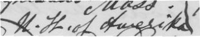U.St. of Amerika.|
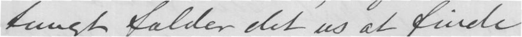tungt falder det os at finde
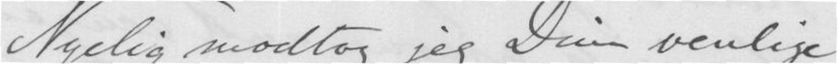Nylig modtog jeg Dine venlige
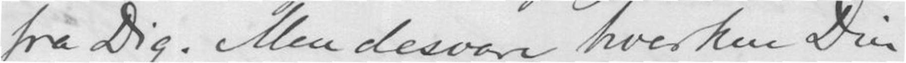fra Dig. Men desvære hverken Din
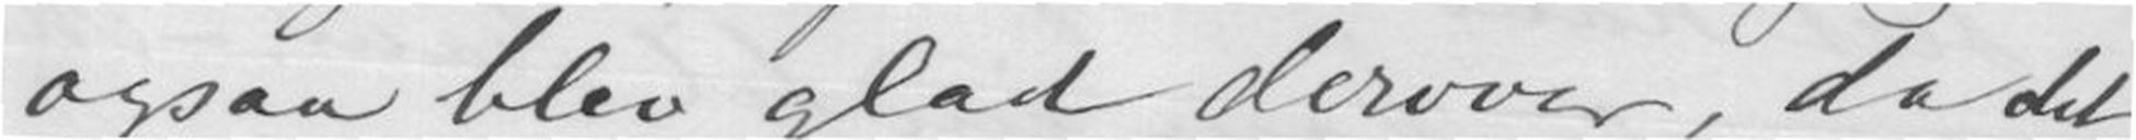ogsaa blev glad derover, da det
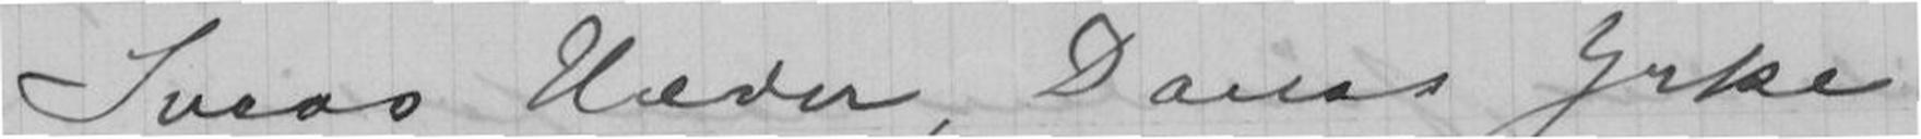"Sveas Hæder, Danas Yrke
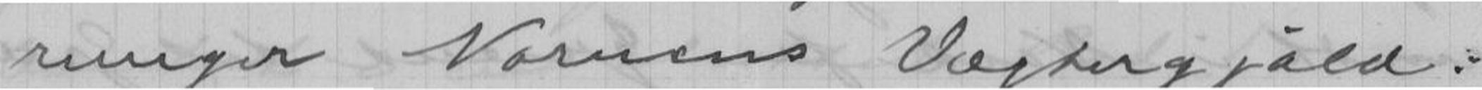ringer Nornens Vægtergjàld:
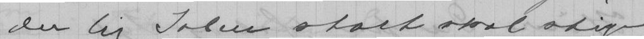der lig Solen stolt skal stige
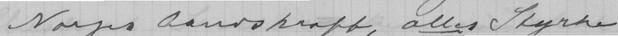Norges Aandskraft, alles Styrke
